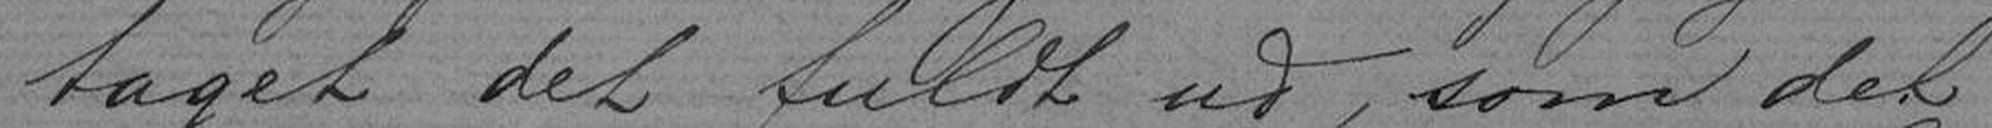taget det fuldt ud, som det
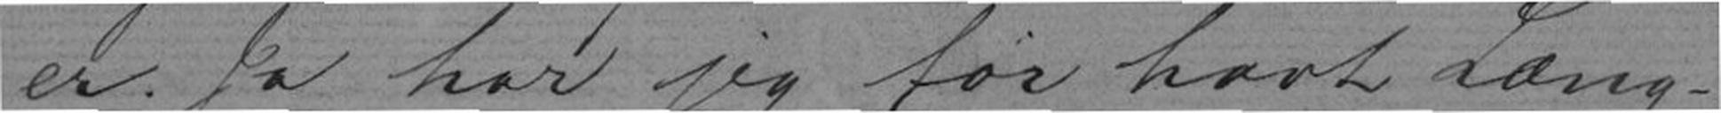er. Ja har jeg før havt Læng-
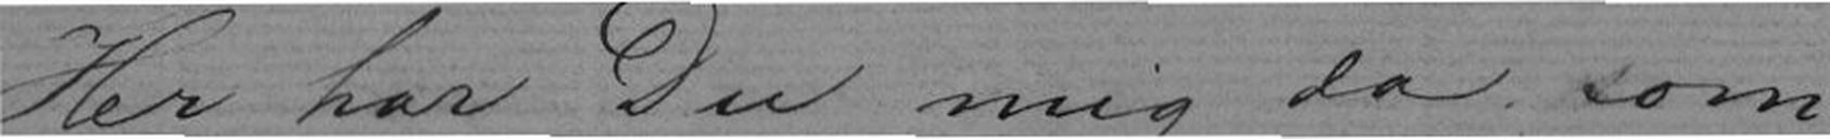Her har Du mig da som
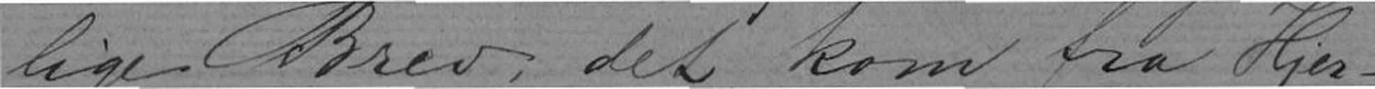lige Brev; det kom fra Hjer-
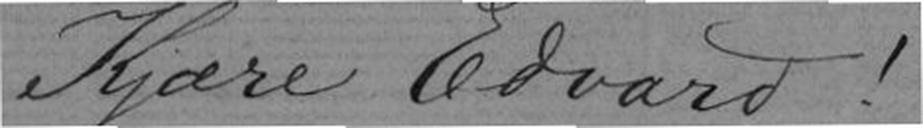Kjære Edvard!
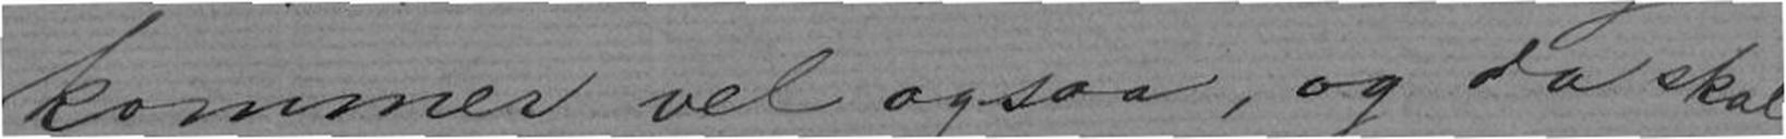kommer vel ogsaa, og da skal
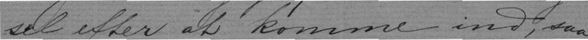sel efter at komme ind, saa
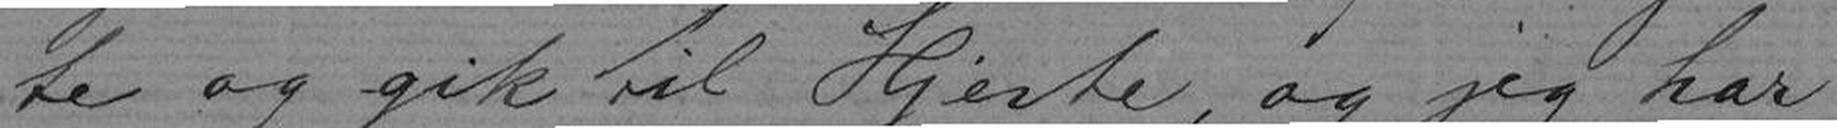te og gik til Hjerte, og jeg har
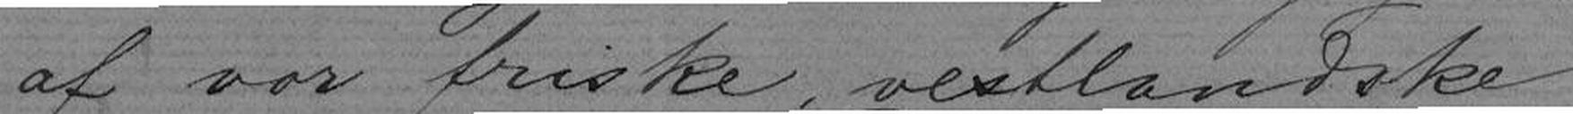af vor friske, vestlandske
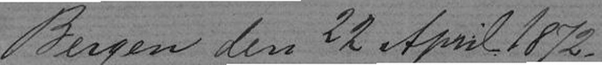Bergen den 22 April 1872.
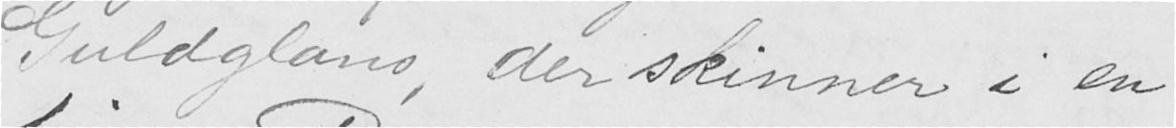Guldglans, der skinner i en
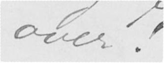over!
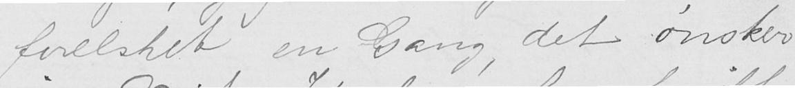forelsket en Gang, det ønsker
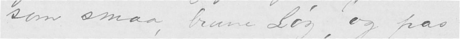som smaa, brune Løg, og pas-
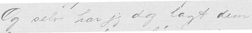Og selv har jeg dog lagt dem
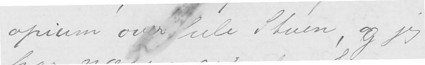opium over hele Stuen, og jeg
